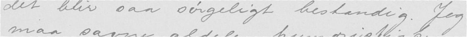det blir saa sørgeligt bestandig. Jeg
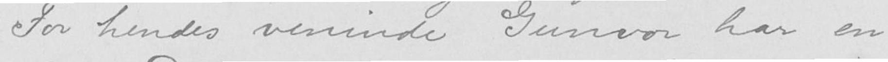For hendes veninde Gunvor har en
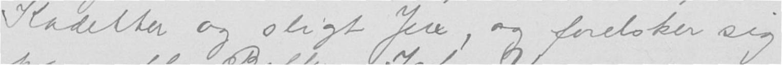Kadetter og sligt Jux og forelsker sig
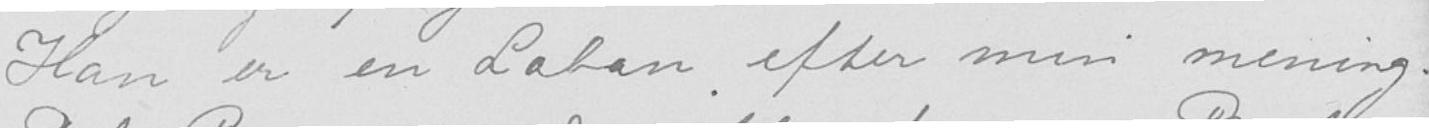Han er en Laban efter min mening.
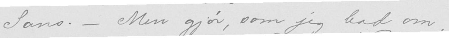Sans. - Men gjør, som jeg bad om
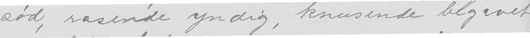sød, rasende yndig, knusende begavet,
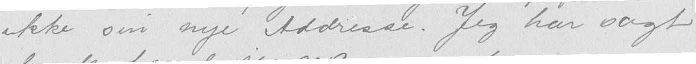ikke sin nye Addresse. Jeg har sagt
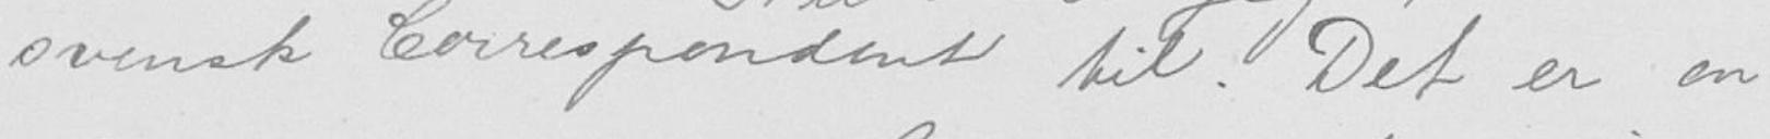svensk Correspondent til! Det er en
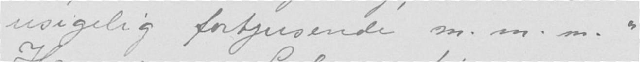usigelig fortjusende m.m.m."
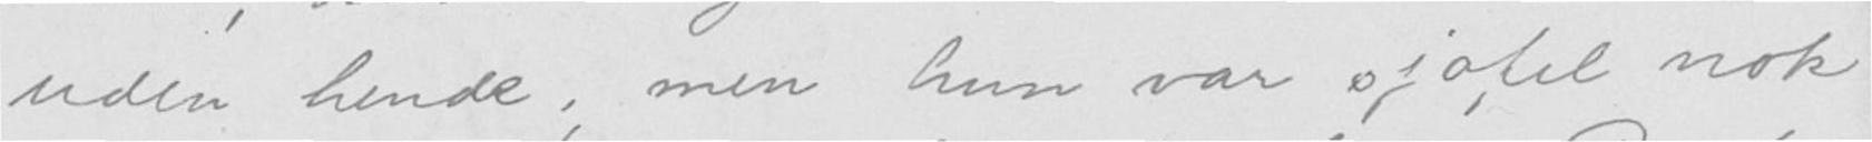uden hende, men hun var sjofel nok
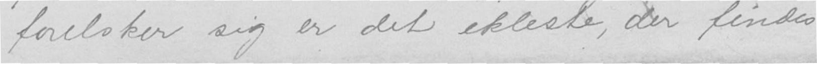forelsker sig er det ekleste, der findes
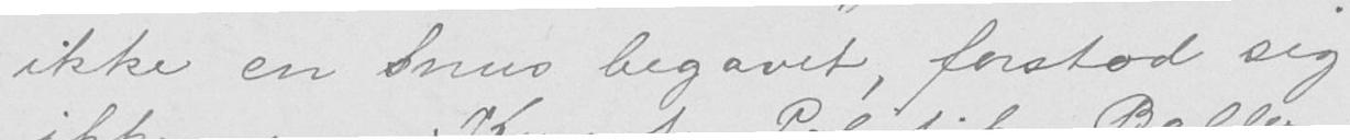ikke en Snus begavet, forstod sig
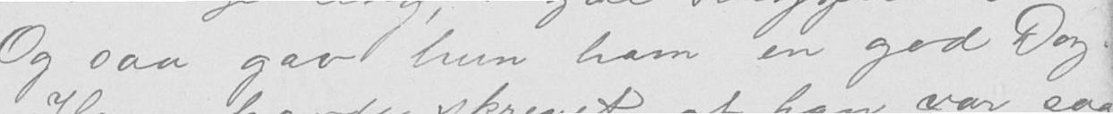Og saa gav hun ham en god Dag.
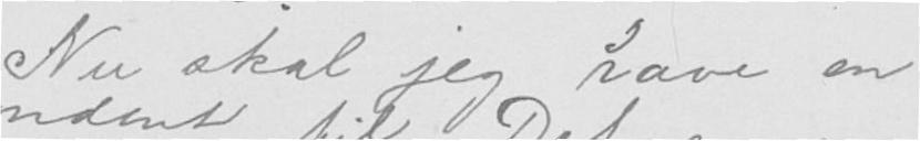Nu skal jeg have en
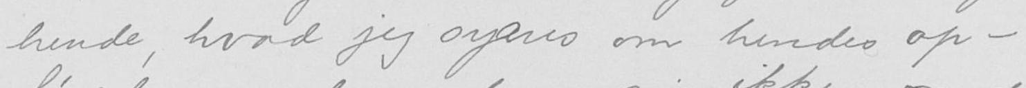hende, hvad jeg synes om hendes op-
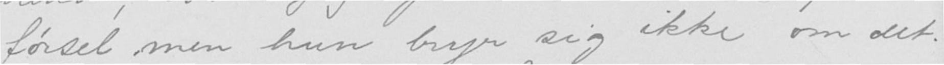førsel, men hun bryr sig ikke om det.
